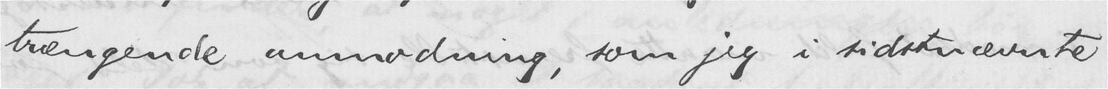trængende anmodning, som jeg i sidstnævnte
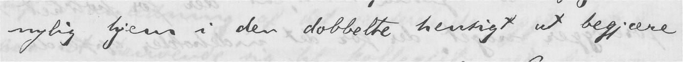nylig hjem i den dobbelte hensigt at begjære
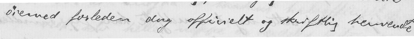øiemed forleden dag officielt og skriftlig henvendte
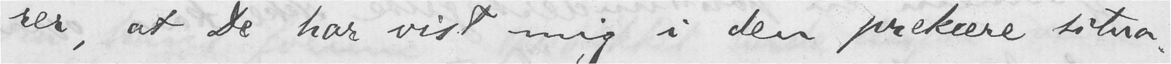rer, at De har vist mig i den prekære situa-
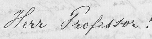Herr Professor!
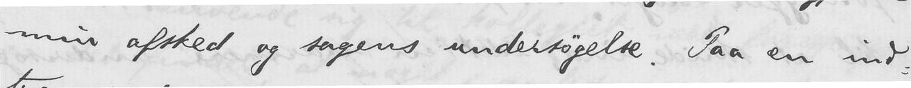min afsked og sagens undersøgelse. Paa en ind-
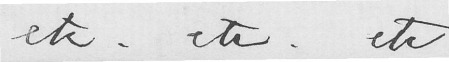etc. etc. etc.
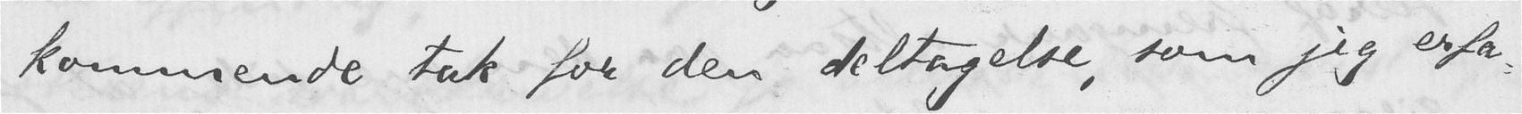kommende tak for den deltagelse, som jeg erfa-
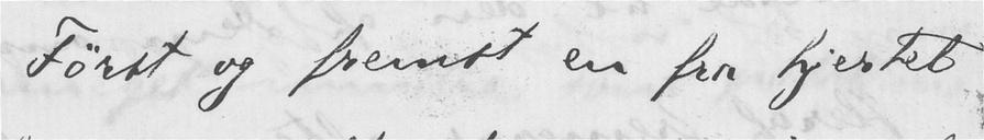Først og fremst en fra hjertet
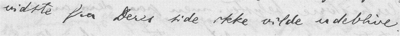vidste fra Deres side ikke vilde udeblive.
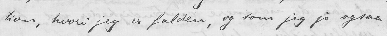tion, hvori jeg er falden, og som jeg jo ogsaa
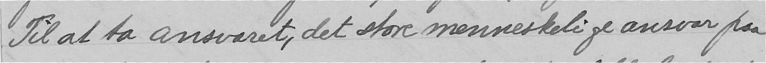Til at ta ansvaret, det store menneskelige ansvar paa
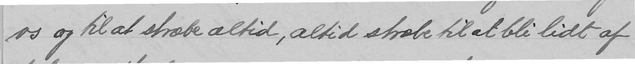os og til at stræbe altid, altid stræbe til at bli lidt af
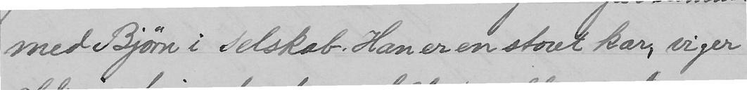med Bjørn i selskab. Han er en stout kar viger
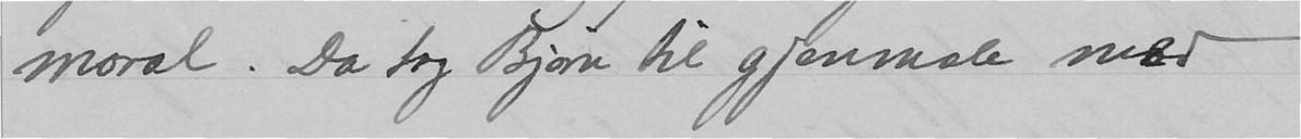moral. Da tog Bjørn til gjenmæle med -
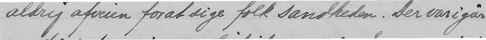aldrig afveien forat sige folk sandheden. Der var igår
